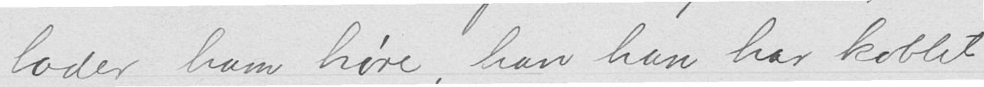lader ham høre, han han har koblet
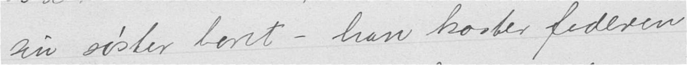sin søster bort - han kaster faderen
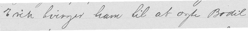Erik tvinger ham til at ægte Bodil
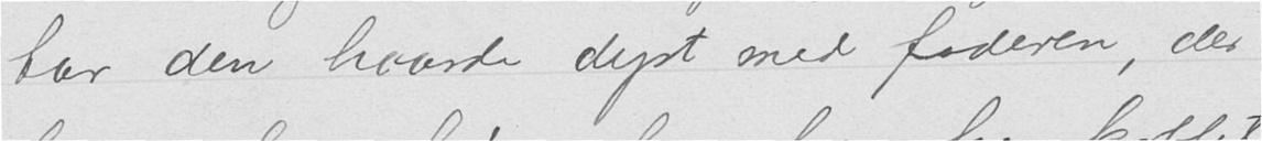tar den haarde dyst med faderen, der
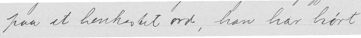paa et henkastet ord, han har hørt
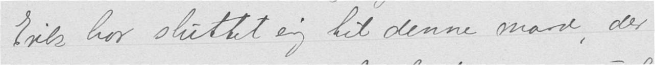Erik har sluttet seg til denne mand, der
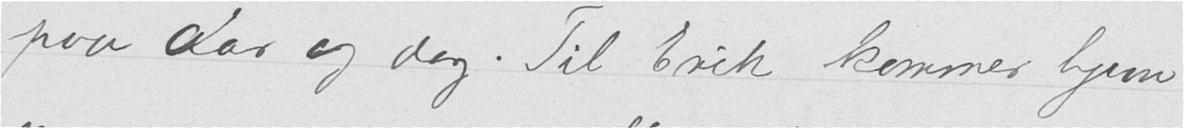paa aar og dag. Til Erik kommer hjem
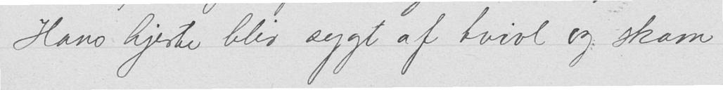Hans hjerte blir sygt af tvivl og skam
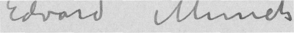Edvard Munch
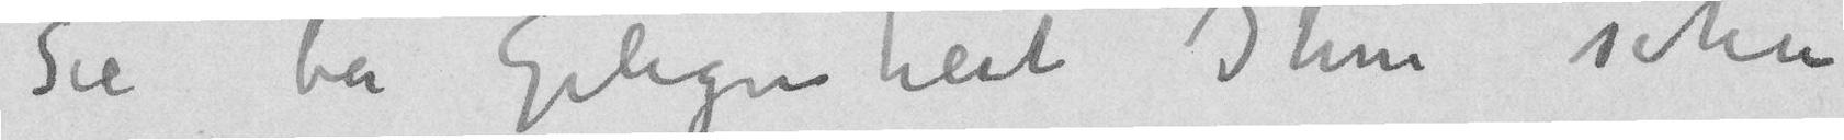Sie bei Gelegenheit Ihm sehen
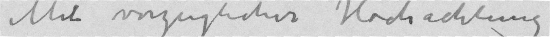Mit vorzuglicher Hochachtung
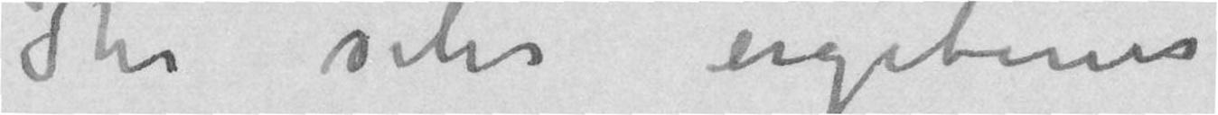Ihr sehr ergebener
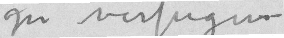zu verfügen
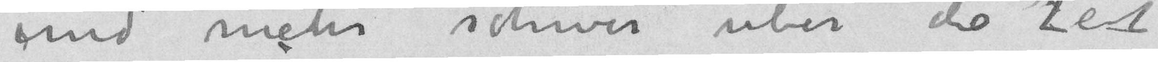und mehr schwer uber die Zeit
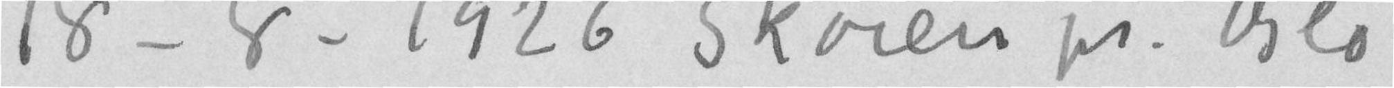18–8–1926 Sköien pr. Oslo
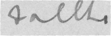sollte
